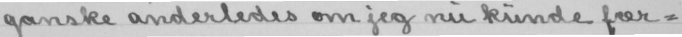ganske anderledes om jeg nu kunde fær-
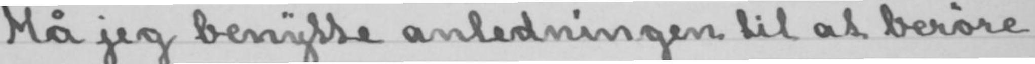Må jeg benytte anledningen til at berøre
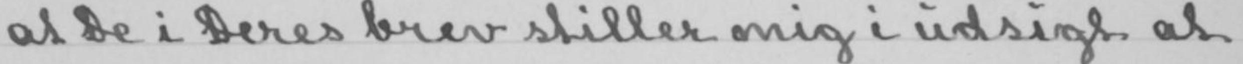at De i Deres brev stiller mig i udsigt at
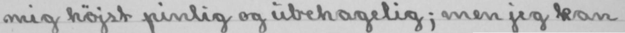mig højst pinlig og ubehagelig; men jeg kan
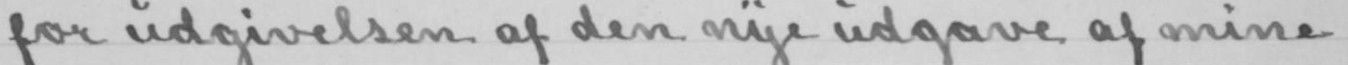for udgivelsen af den nye udgave af mine
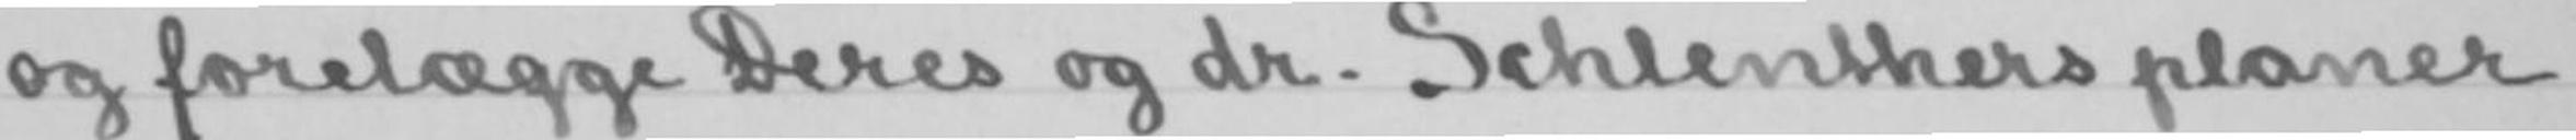og forelægge Deres og dr. Schlenthers planer
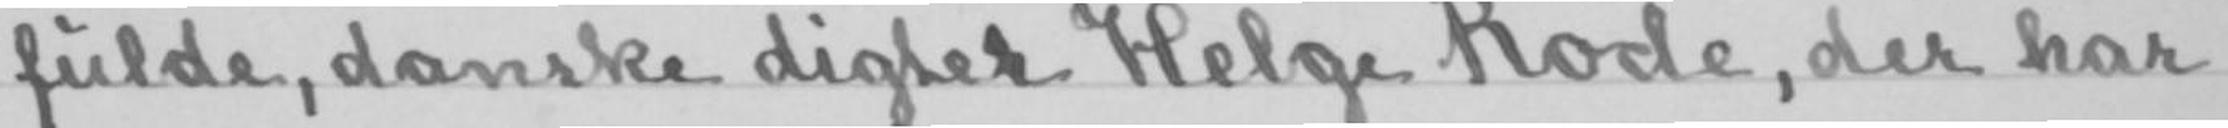fulde, danske digter Helge Rode, der har
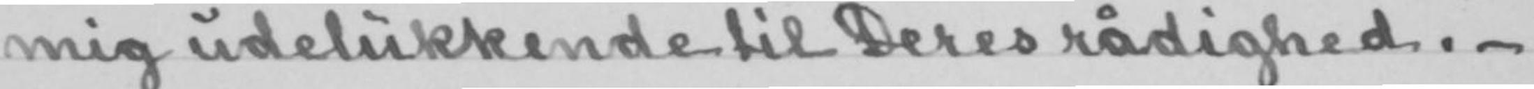mig udelukkende til Deres rådighed.-
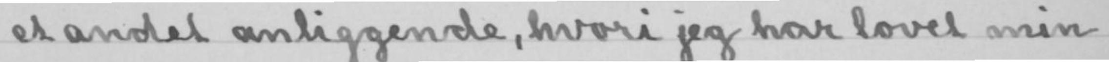et andet anliggende, hvori jeg har lovet min
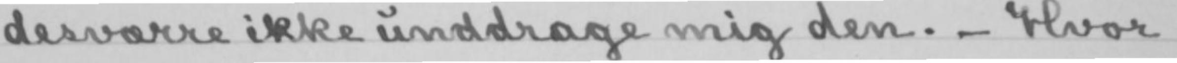desværre ikke unddrage mig den.- Hvor
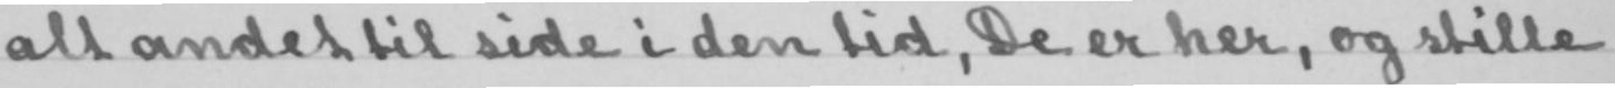alt andet til side i den tid, De er her, og stille
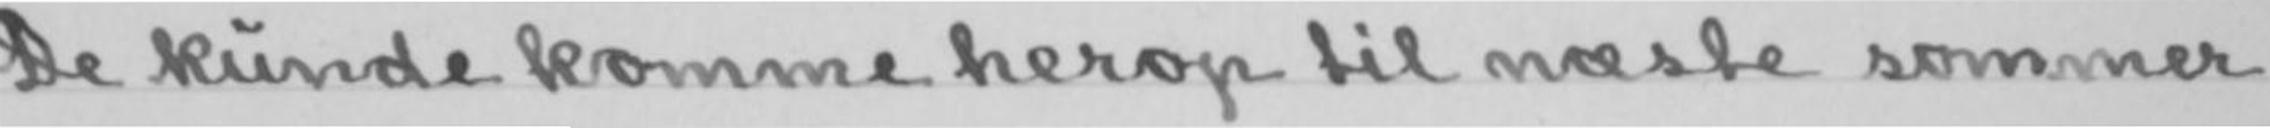De kunde komme herop til næste sommer
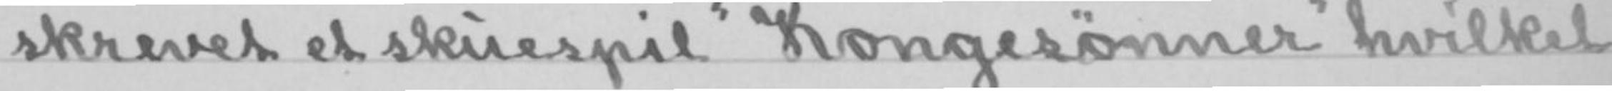skrevet et skuespil «Kongesønner» hvilket
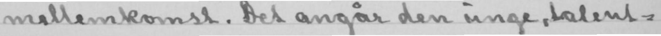mellemkomst. Det angår den unge, talent-
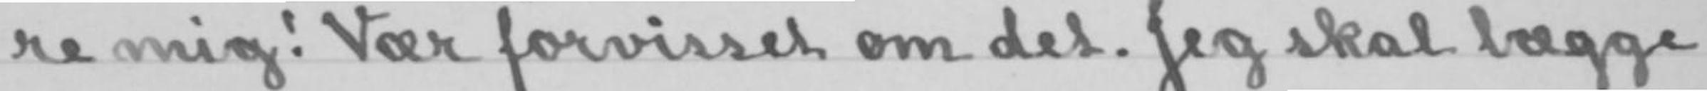re mig! Vær forvisset om det. Jeg skal lægge
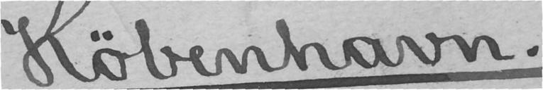København.
